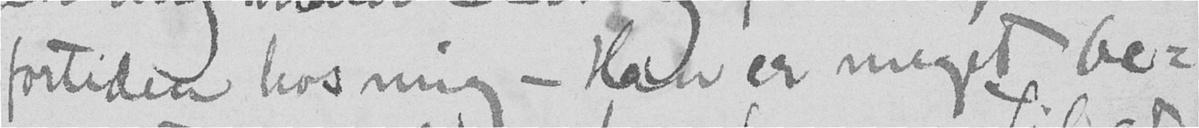fortiden hos mig - Han er meget "be-
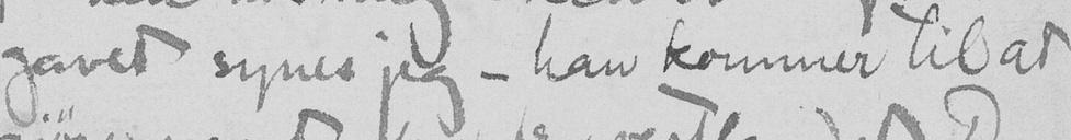gavet synes jeg - han kommer til at
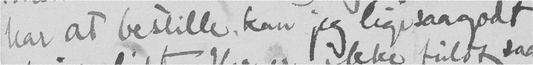har at bestille, kan jeg ligesaagodt
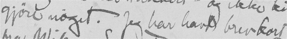gjøre noget. Jeg har havd brevkort
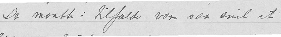De maatte i tilfælde være saa snil at
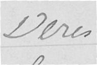Din
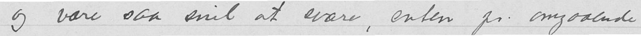og være saa snil at svare, enten pr. omgaaende
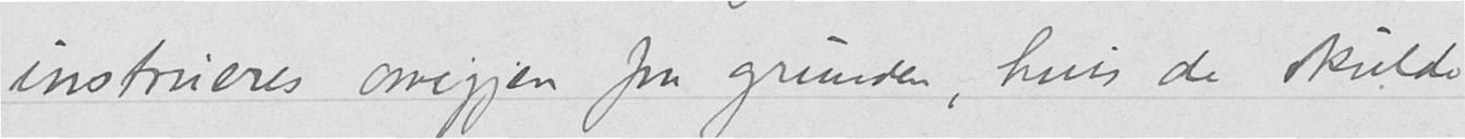instrueres omigjen fra grunden, hvis de skulde
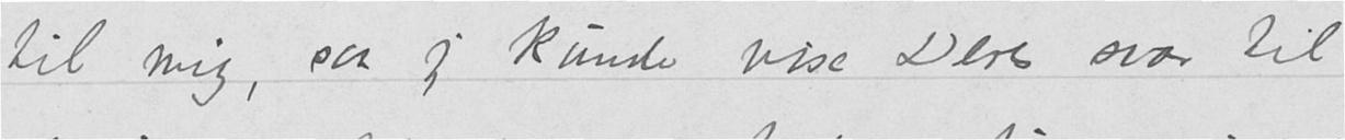til mig, saa jeg kunde vise Deres svar til
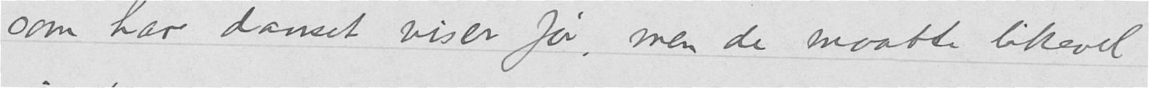som har danset viser før, men de maatte likevel
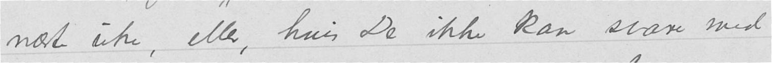næste uke, eller, hvis De ikke kan svære med
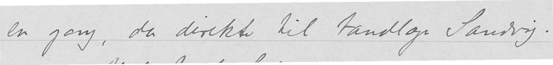en gang, da direkte til tandlæge Sandvig.
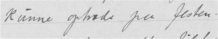kunne optræde paa festen.
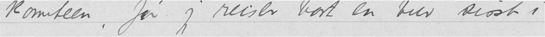komiteen, før jeg reiser bort en tur sisst i
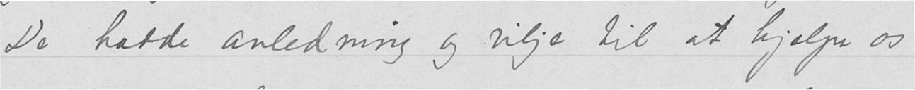De hadde anledning og vilje til at hjelpe os
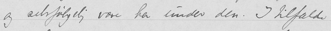og selvfølgelig være her under den. I tilfælde
